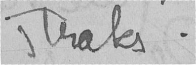straks.
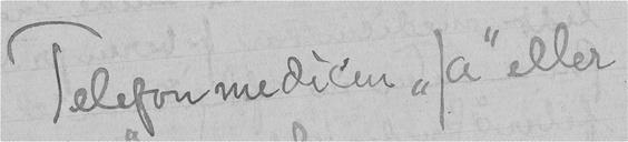Telefon medicin "ja" eller
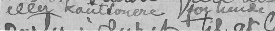eller kautionere for hende
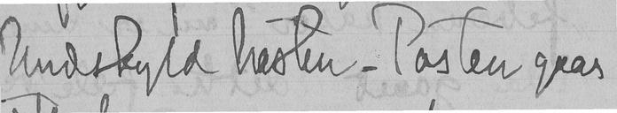Undskyld hasten - Posten gaar
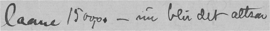laane 1500,00 - nu blir det altsaa
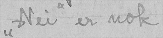"Nei" er nok
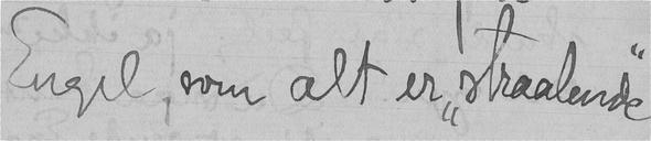Engel, som alt er "straalende".
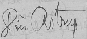Din Astrup
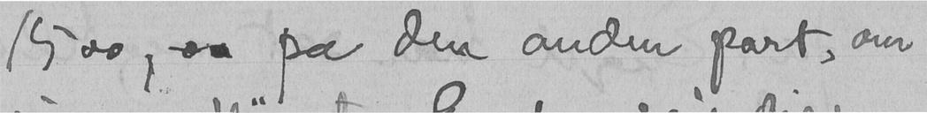1500,00 pa den anden part, om
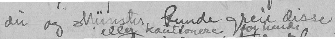du og Münster kunde greie disse
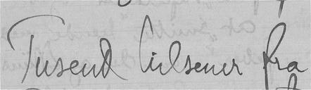Tusend hilsener fra
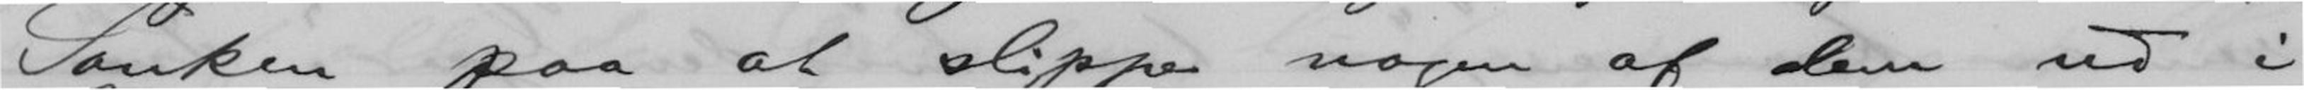Tanken paa at slippe nogen af dem ud i
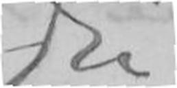Din
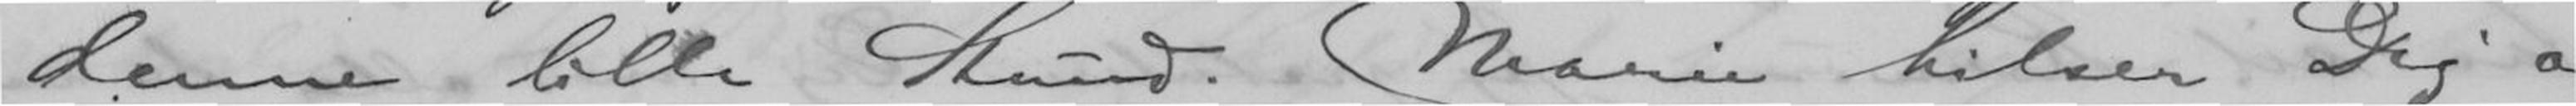denne lille Stund. Marie hilser Dig og
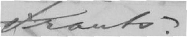Frants.
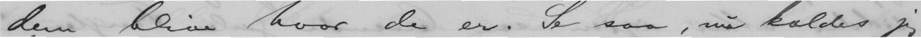dem blive hvor de er. Se saa, nu kaldes jeg
